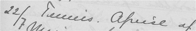22/7 Tennis. Afreise af
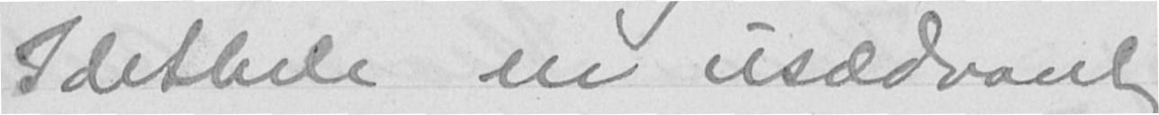Idethele en usædvanlig
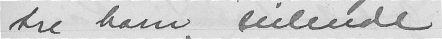tre barn seilende
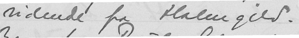ridende fra Holmgild.
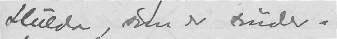Hulda, som er sønder-
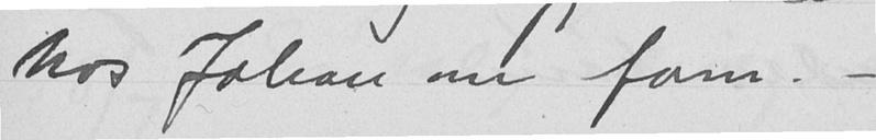hos Johan om form. -
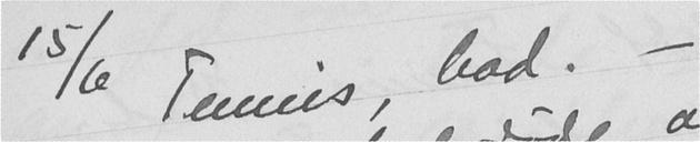15/6 Tennis, bad. -
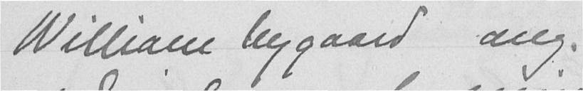William Nygaard ang.
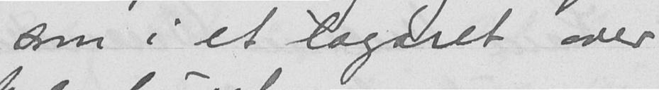som i et lazaret over
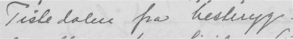Tistedalen fra hesteryg.
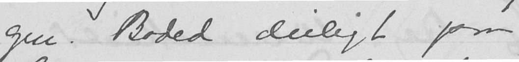gen. Baded deiligt paa
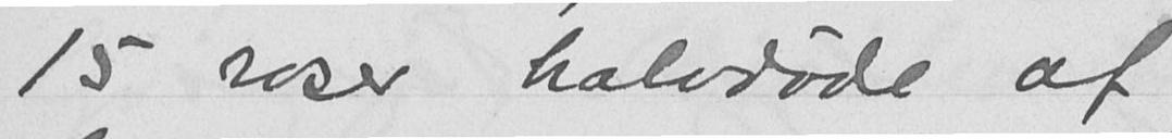15 roser halvdøde af
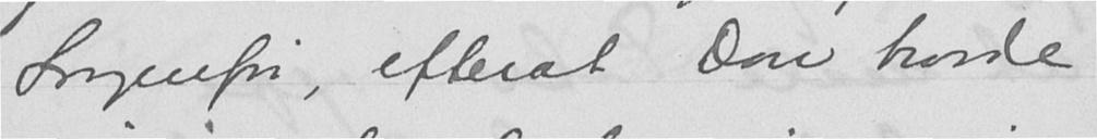Sorgenfri, efterat Don havde
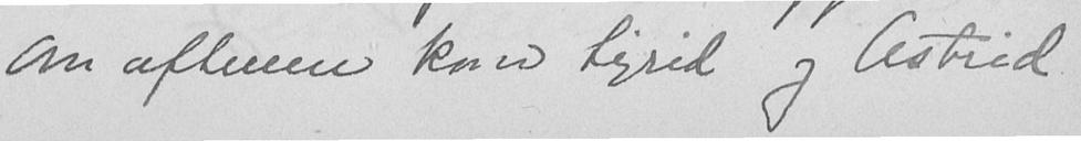Om aftenen kom Sigrid og Astrid
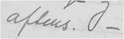aftens. -
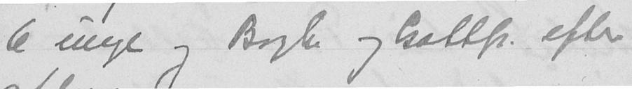6 unge og Borgh. og Gottfr. efter
